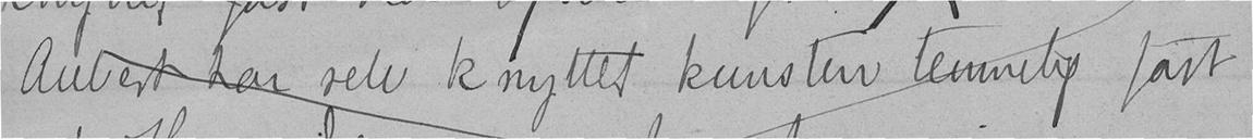Aubert har selv knyttet kunsten temmelig fast
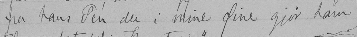fra hans Pen der i mine Øine gjør ham
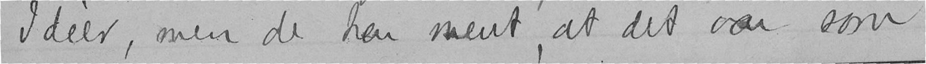Idéer, men de har ment, at det var som
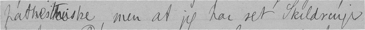pathesthiske, men at jeg har set Skildringer
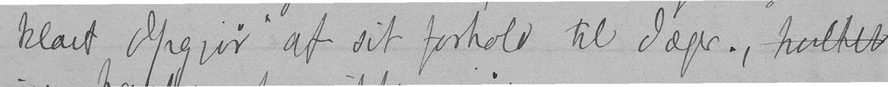klart Opgjør af sit forhold til Jæger., hvilket
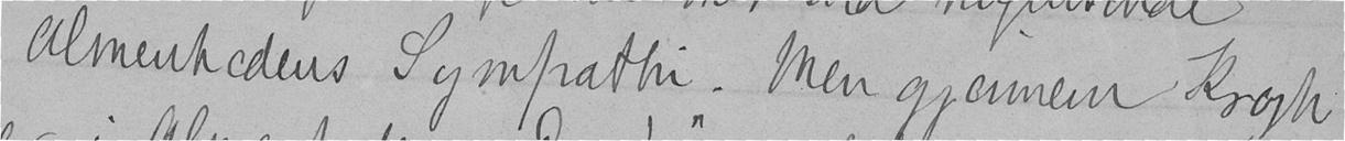Almenhedens Sympathi. Men gjennem Krogh
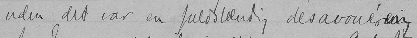uden det var en fuldstændig desavouéring
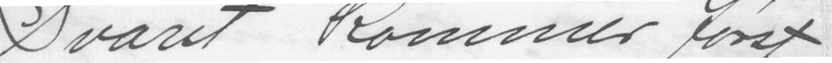Svaret kommer først
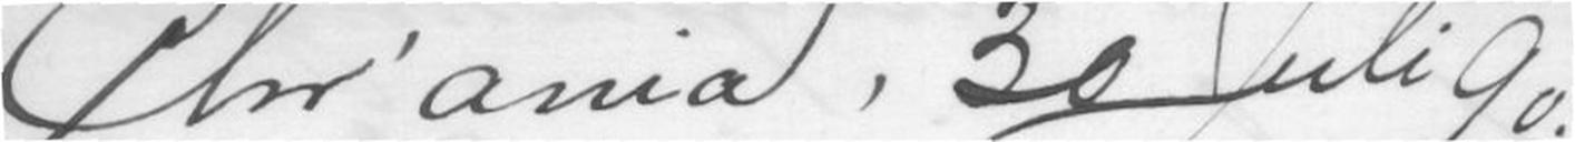Chr'ania, 30 Juli 90.
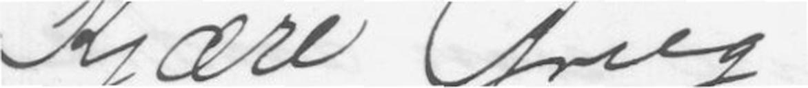Kjære Grieg!
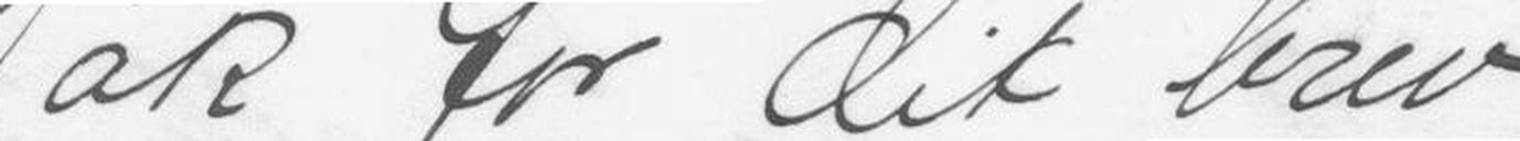Tak for dit brev.
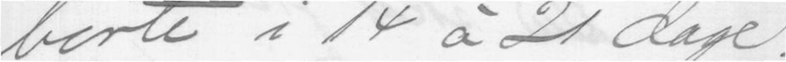borte i 14 à 21 dage.
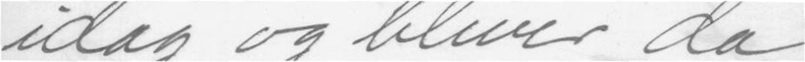idag og bliver da
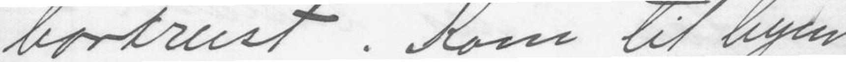bortreist. Kom til byen
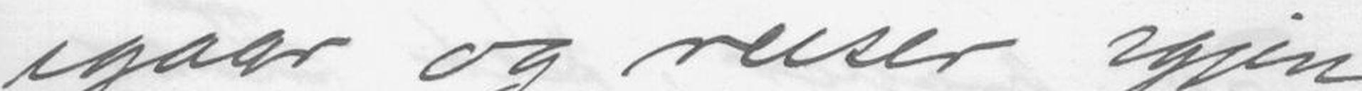igaar og reiser igjen
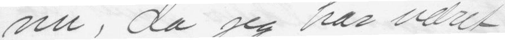nu, da jeg har været
